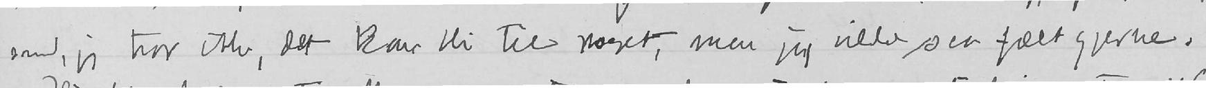med, jeg tror ikke, det kan bli til noget, men jeg vilde saa fælt gjerne.
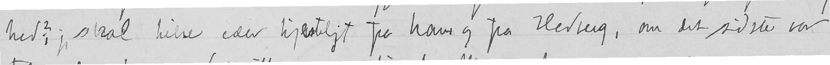hed?, jeg skal hilse eder hjerteligt fra ham og fra Hedberg, om det sidste var
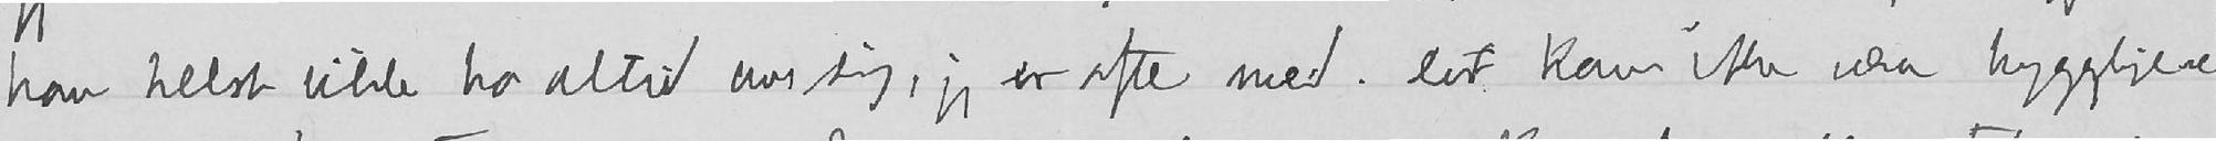han helst vilde ha altid hos sig, jeg er ofte med. Det kan ikke være hyggeligere
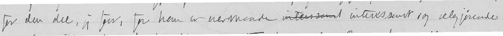for den del, jeg faar, for han er overmaade interssant interessant og velgjørende
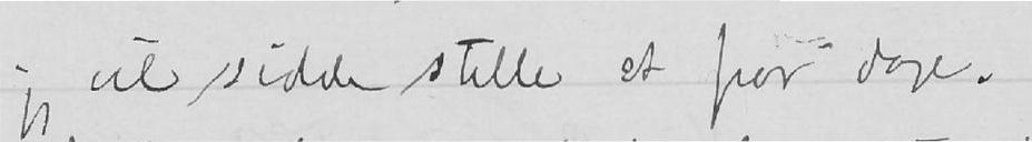jeg vil sidde stille et par dage.
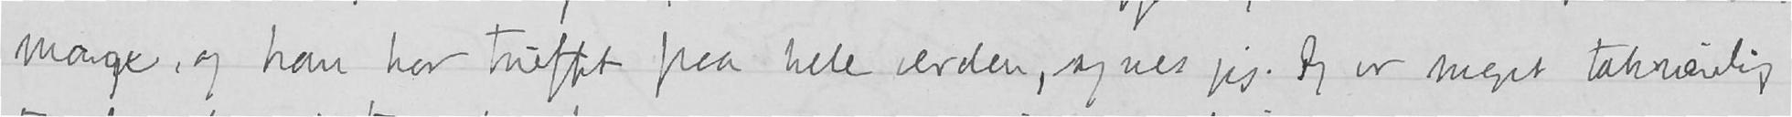mange, og han har truffet paa hele verden, synes jeg. Jeg er meget taknemlig
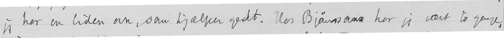jeg har en liden ovn, som hjælper godt.Hos Bjørnsons har jeg vært to gange,
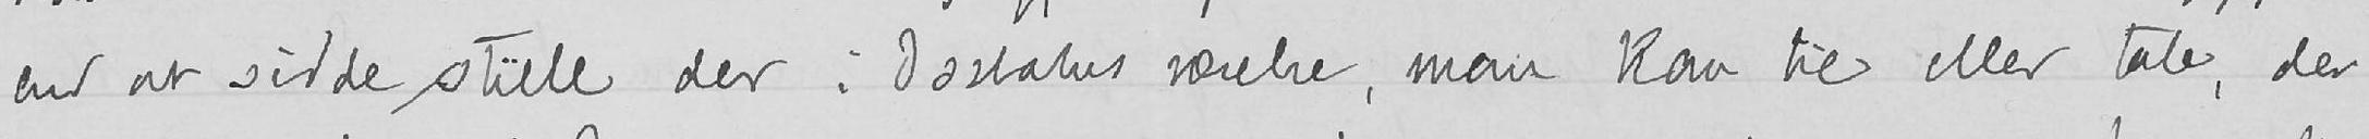end at sidde stille der i Ossbahrs værelse, man kan tie eller tale, der
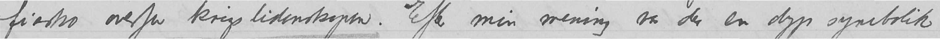fiasko overfor krigslidenskapen.  Efter min mening var der en dyp symbolik
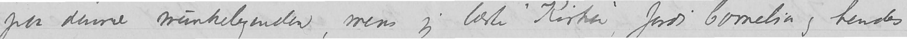på denne munkelegenden, mens jeg læste «Kirken», fordi Cornelia og hendes
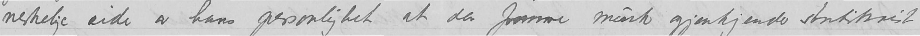neskelige side av hans personlighet at den fromme munk gjenkjender Antikrist.
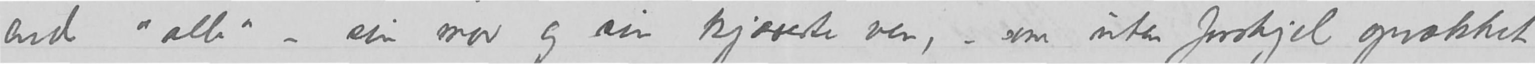end «alle» – sin mor og sin kjæreste ven, – som uten forskjel opvækket
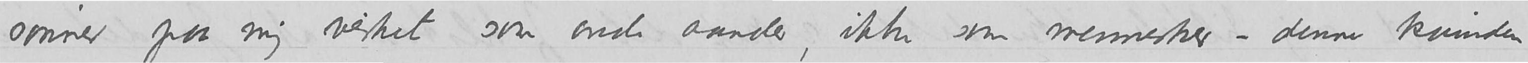sønner paa mig virket som onde aander, ikke som mennesker – denne kvinden
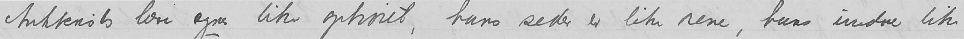Antikrists lære synes  like ophøiet, hans seder er like rene, hans undre like
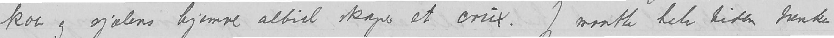kaar og sjælens hjemve altid skaper et crux.  Jeg maatte hele tiden tenke
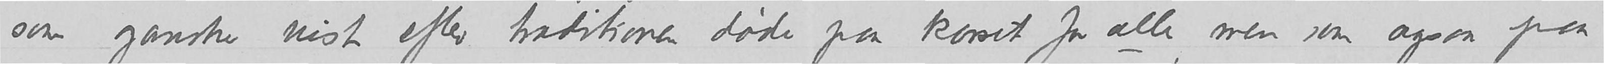efter traditionen døde på korset for alle, men som ogsaa paa
